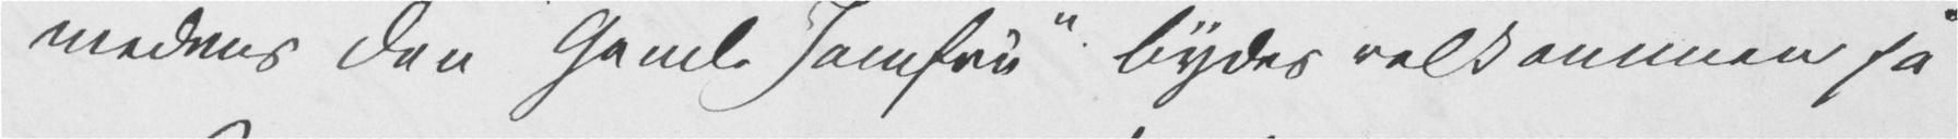medens den "Gamle Jomfru" bydes velkommen på
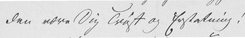den være Dig Trøst og Erstatning!
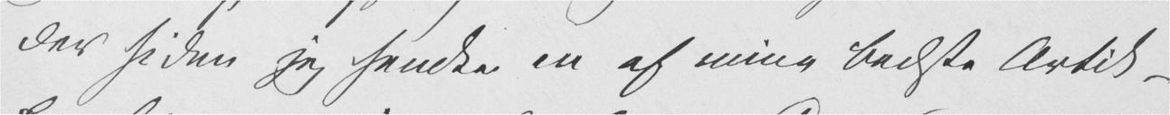der siden jeg sendte en af mine bedste Artik-
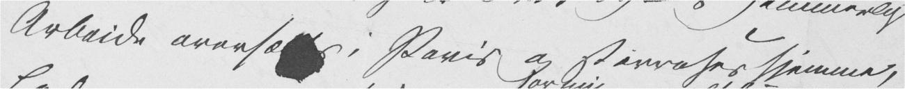Arbeide oversættes i Paris og storroses hjemme,
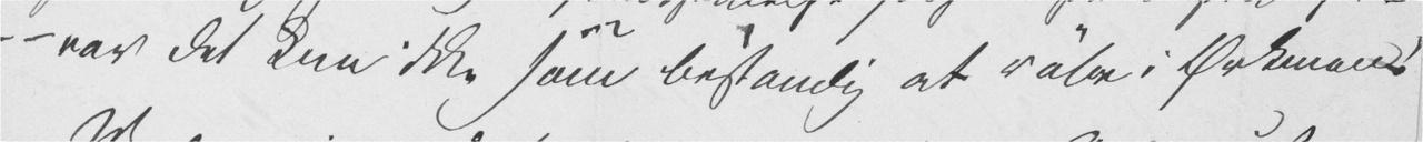var det kun ikke som bestandig at råbe i Ørkenen!
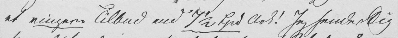et ringere Tilbud end 7 1/2 Spd Ark! Jeg sender Dig
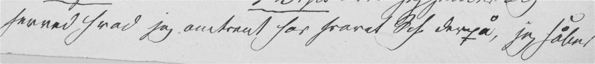herved hvad jeg omtrent har svaret Sch. derpå, jeg håber
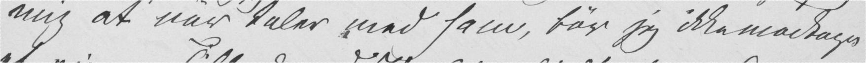mig at når taler med ham, tør jeg ikke modtage
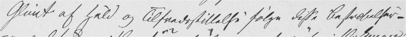Glimt af Held og Tilfredsstillelse følge disse Bestræbelser –
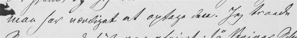man har værdiget at optage den. Jeg troede
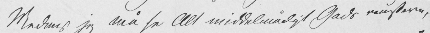Medens jeg må se Alt middelmådigt Gods renstere,
